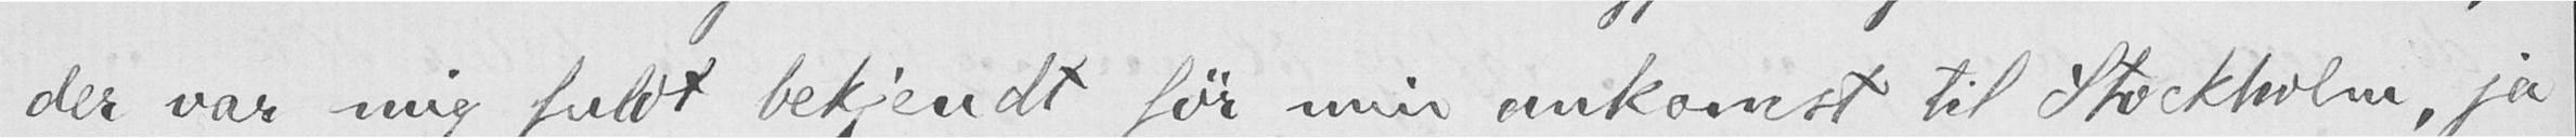der var mig fuldt bekjendt før min ankomst til Stockholm, ja
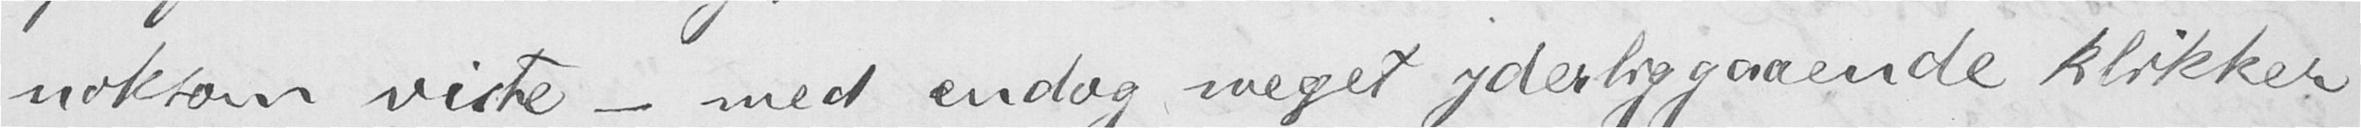noksom viste - med endog meget yderliggaaende klikker
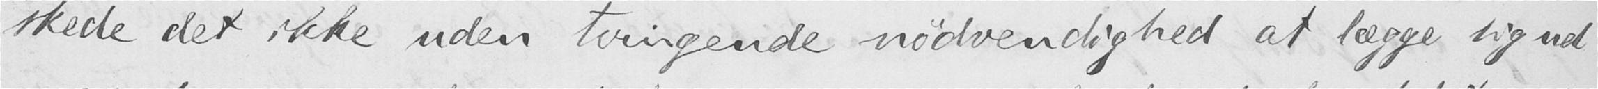skede det ikke uden tvingende nødvendighed at lægge sig ud
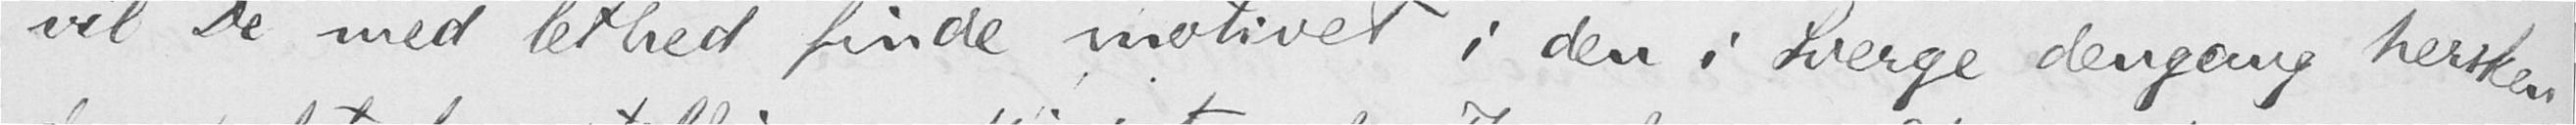vil De med lethed finde motivet i den i Sverge dengang hersken-
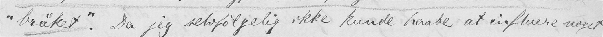"bråket". Da jeg selvfølgelig ikke kunde haabe at influere noget
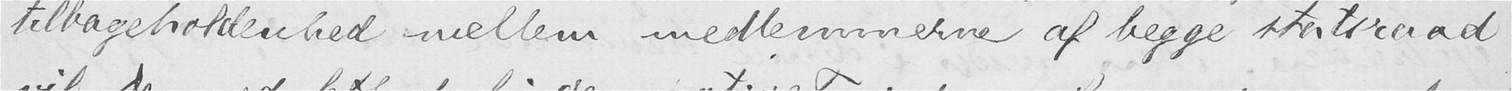tilbageholdenhed mellem medlemmerne af begge statsraad
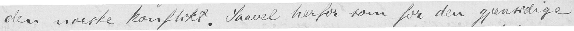den norske konflikt. Saavel herfor som for den gjensidige
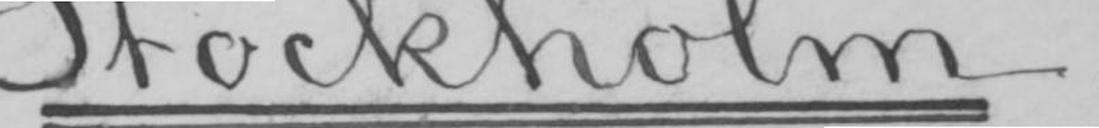Stockholm.
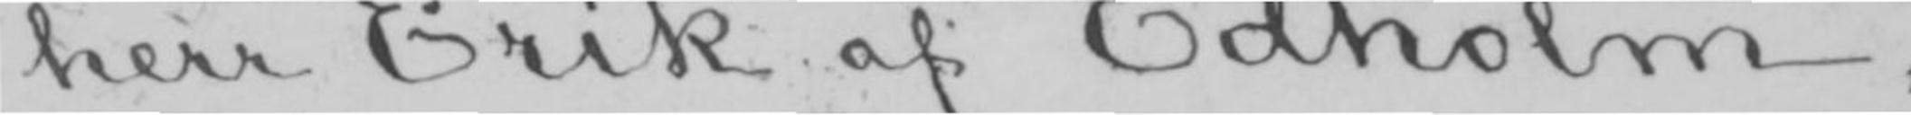herr Erik af Edholm,
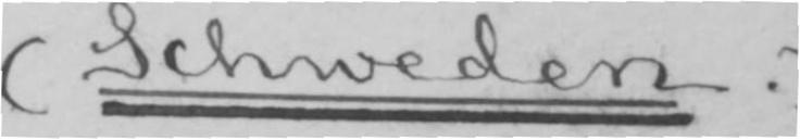(Schweden.)
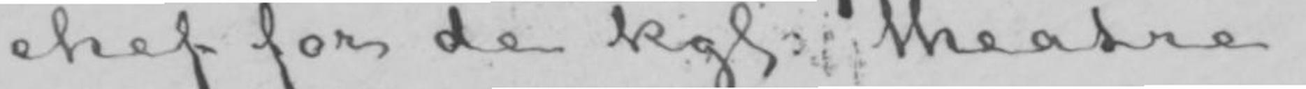chef for de kgl: theatre.
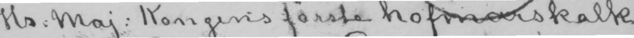Hs: Maj: Kongens første hofmarskalk
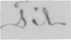Til
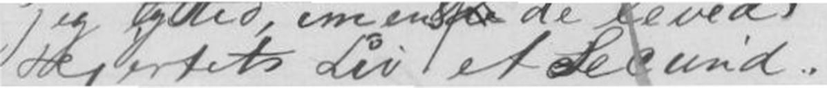Hjertets Liv et Secund.
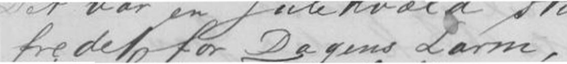fredet for Dagens Larm,
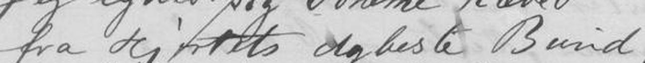fra Hjertets dybeste Bund,
